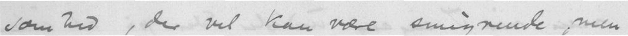somhed, der vel kan være smigrende, men
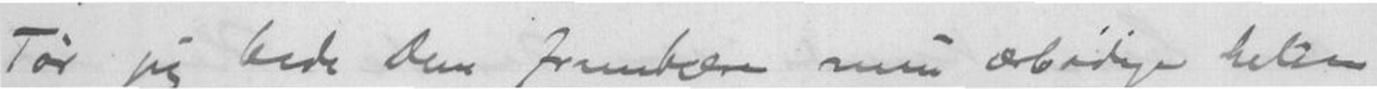Tør jeg bede Dem frembære min æbødige hilsen
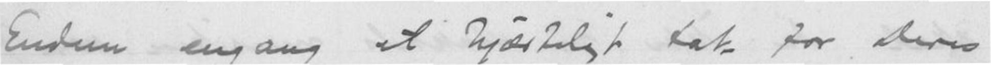Endnu engang et hjærteligt tak for Deres
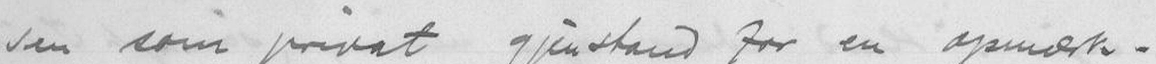sen som privat gjenstand for en opmærk-
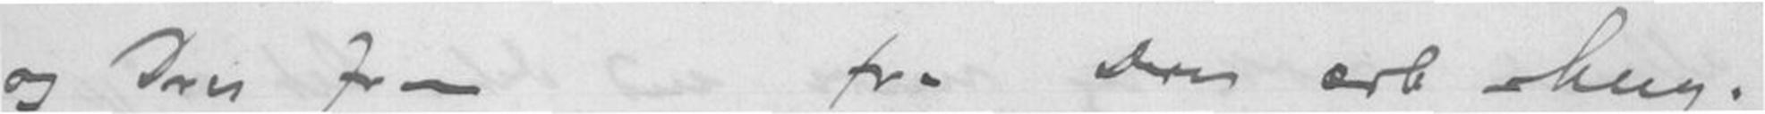og Deres frufra Deres ærb. heng.
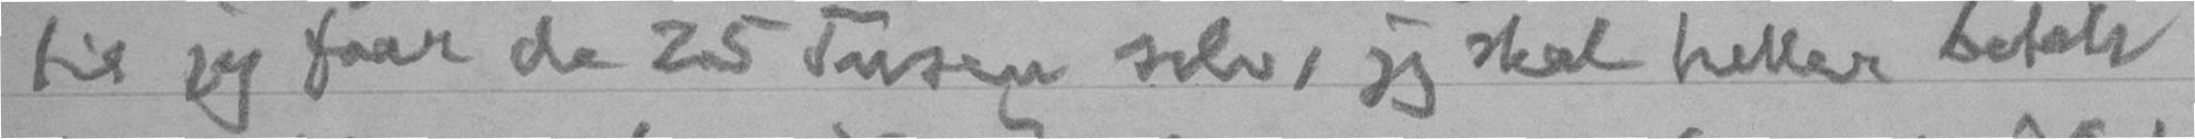til jeg faar de 25 Tusen selv, jeg skal heller betale
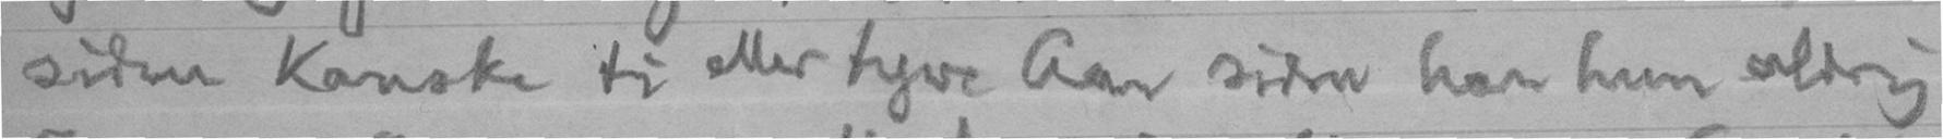siden kanske ti eller tyve Aar siden har hun aldrig
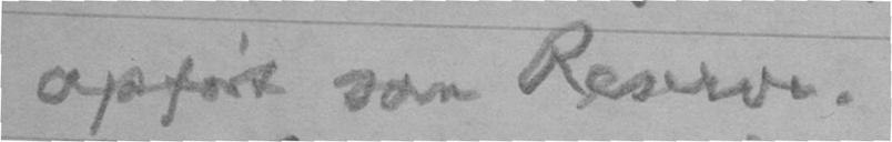opført som Reserve.
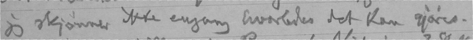jeg skjønner ikke engang hvorledes det kan gjøres.
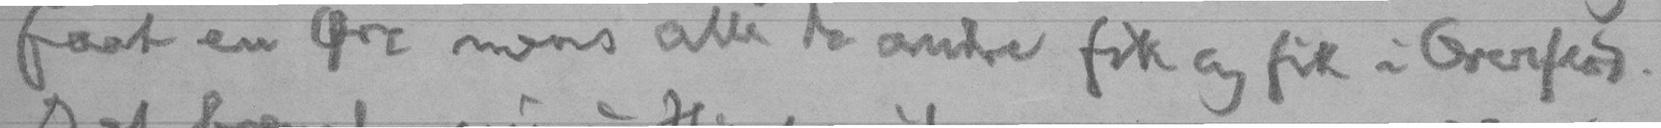faat en Øre mens alle de andre fik og fik i Overflod.
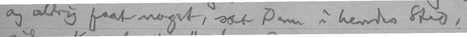og aldrig faat noget, sæt Dem i hendes Sted,
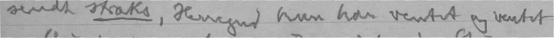sendt straks, Herregud hun har ventet og ventet
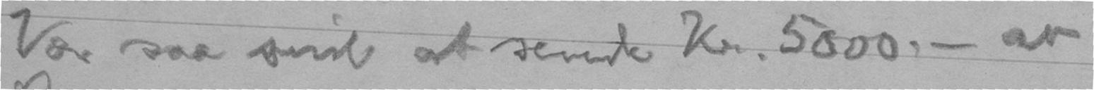Vær saa snil at sende Kr. 5000. - av
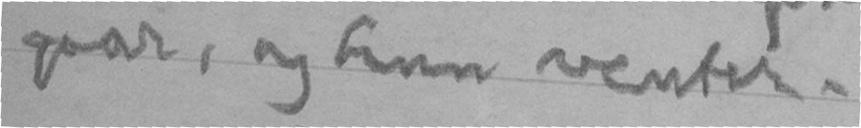gaar, og hun venter.
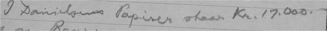I Danielsens Papirer staar Kr. 17.000. -
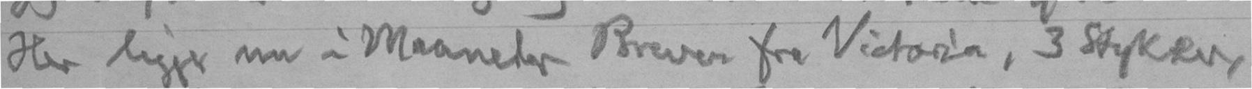Her ligger nu i Maaneder Brever fra Victoria, 3 Stykker,
